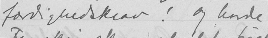færdighedskrav! Og havde
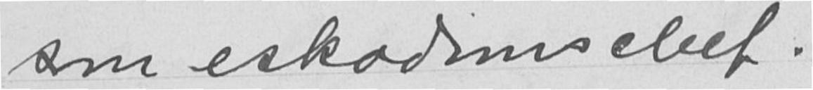som eskadronschef.
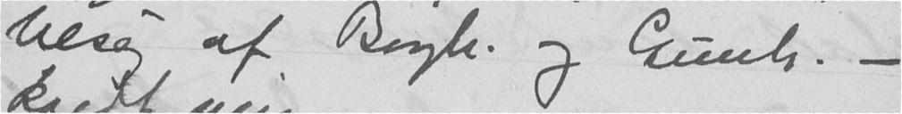besøg af Borgh. og Gunh. -
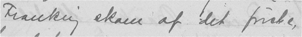Frankrig skam af det første,
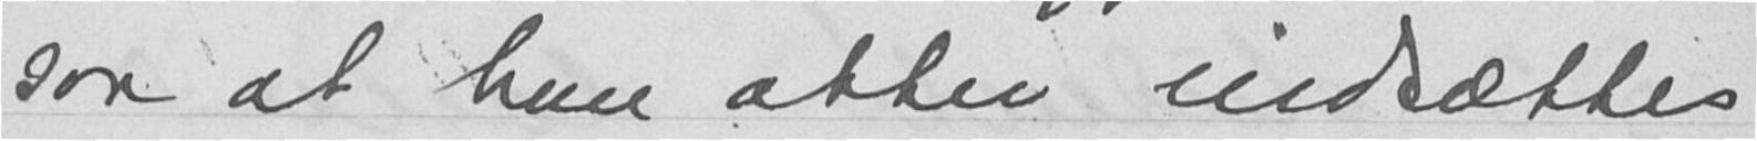saa at han atter indsættes
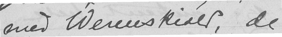med Werenskiold, de
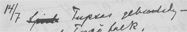14/7 Spiste Tupsas geburtsdag -
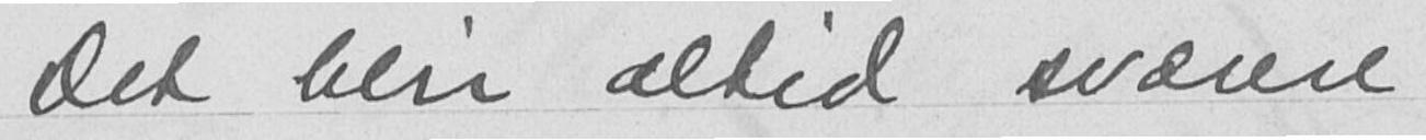Det blir altid sværere
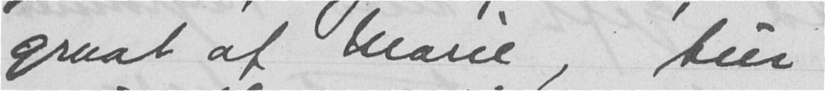graat af Marie, tur
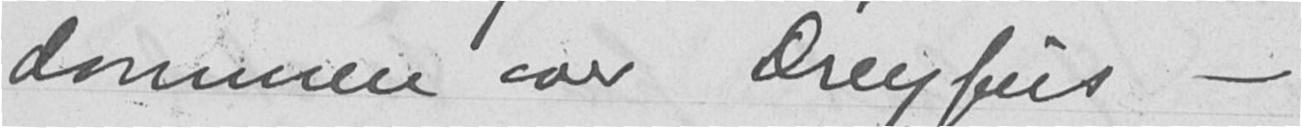dommen over Dreyfus -
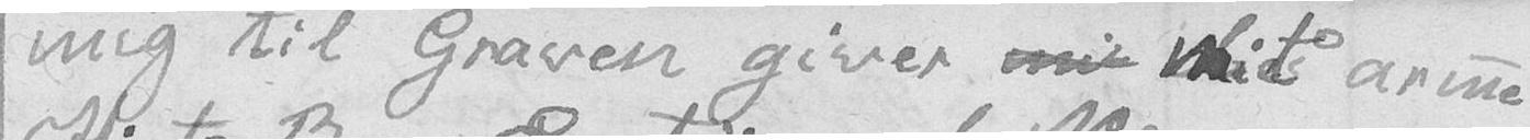mig til Graven giver mi mit arme
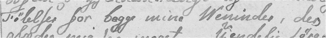Følelser for begge mine Veninder, der
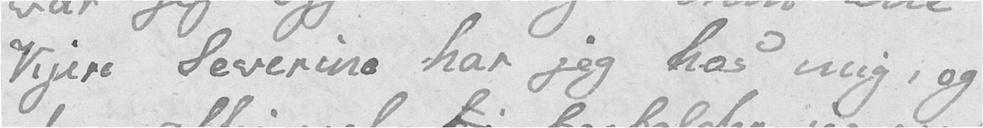kjere Severine har jeg hos mig, og
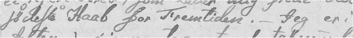sødeste Haab for Fremtiden. – Jeg er i
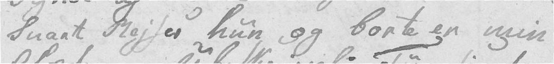Snart Rejser hun og borte er min
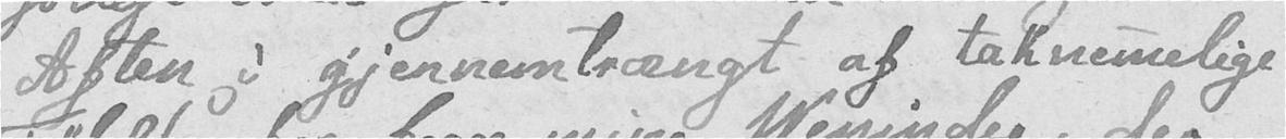Aften i gjennemtrængt af taknemmelige
